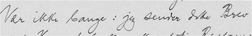Vær ikke bange: jeg sender dette Brev
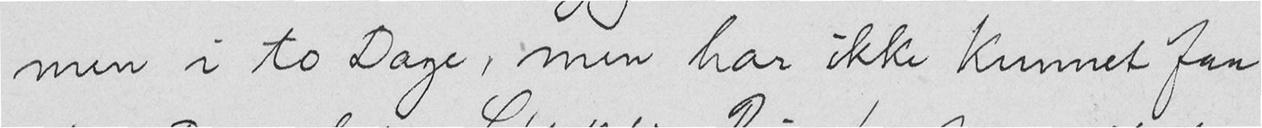men i to Dage, men har ikke kunnet faa
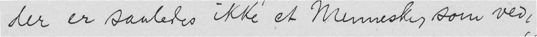der er saaledes ikke et Menneske, som ved,
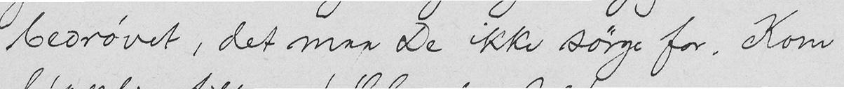bedrøvet, det maa De ikke sørge for. Kom
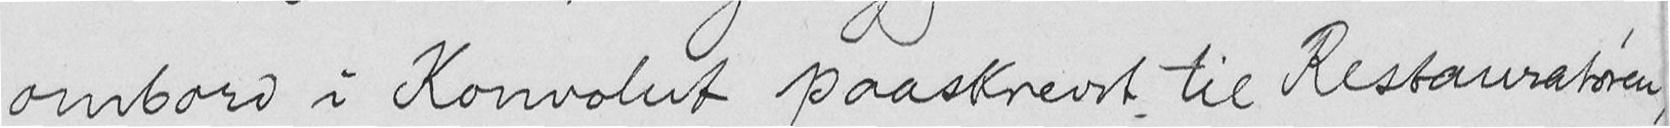ombord i Konvolut paaskrevet til Restauratøren,
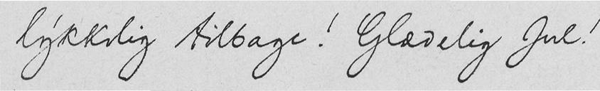lykkelig tilbage! Glædelig Jul!
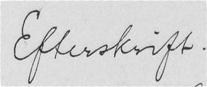Efterskrift.
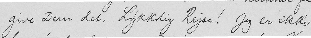give Dem det. Lykkelig Rejse! Jeg er ikke
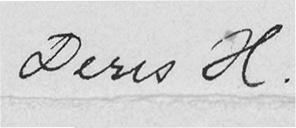Deres H.
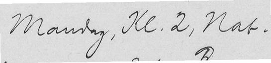Mandag, Kl. 2, Nat.
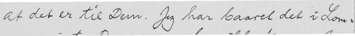at det er til Dem. Jeg har baaret det i Lom-
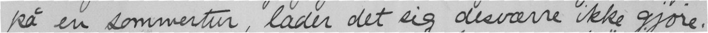på en sommertur, lader det sig desværre ikke gjøre.
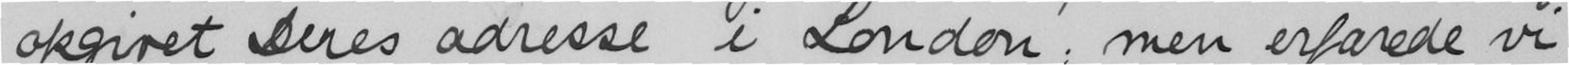opgivet Deres andresse i London; men erfarede vi
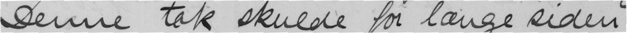Denne tak skulde for længe siden
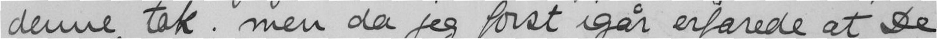denne tak; men da jeg først igår erfarede at De
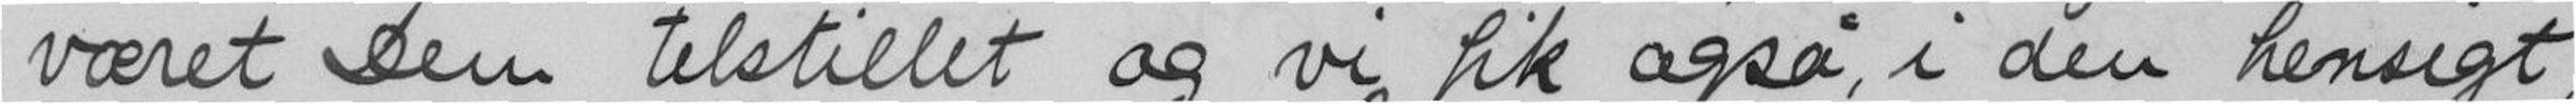været Dem tilstillet og vi fik også, i den hensigt,
